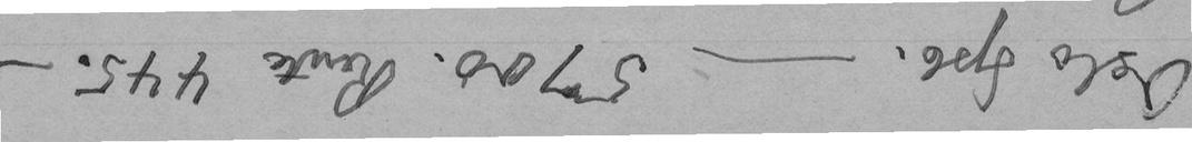Oslo Spb. - 5700. Rente 445. -
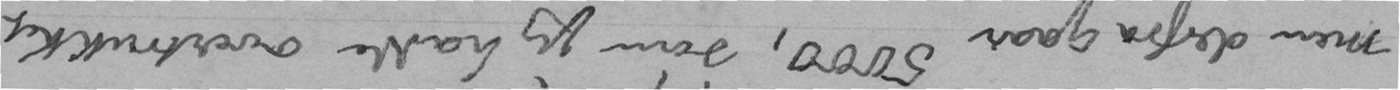men derfra gaar 5000, som jeg hadde overtrukket
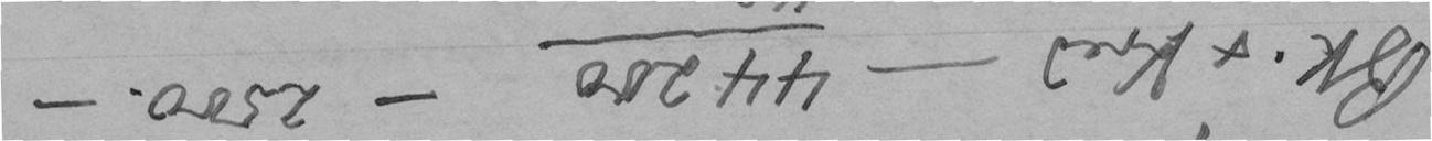BK. x Kred - 44 200 - 2500. -
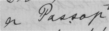er Passop
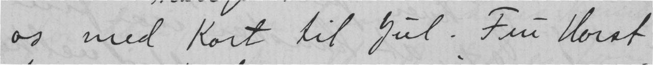os med Kort til jul. Fnu Horst
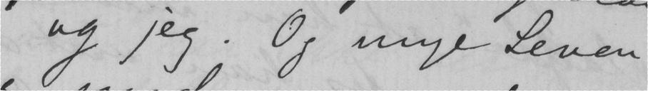og jeg. Og mye Leven
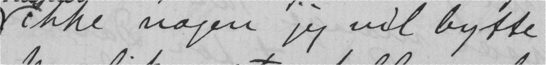ikke nogen jeg vil bytte
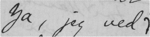Ja, jeg ved
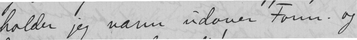holder jeg varm udover Form. og
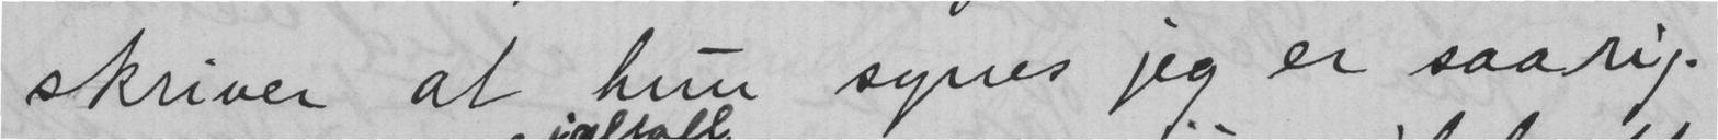skriver at hun synes jeg er saa rig.
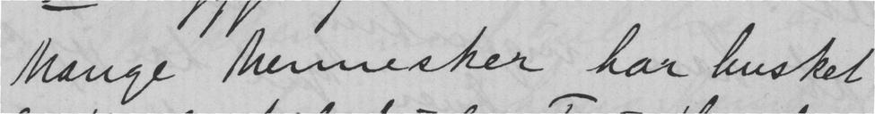Mange mennesker har husket
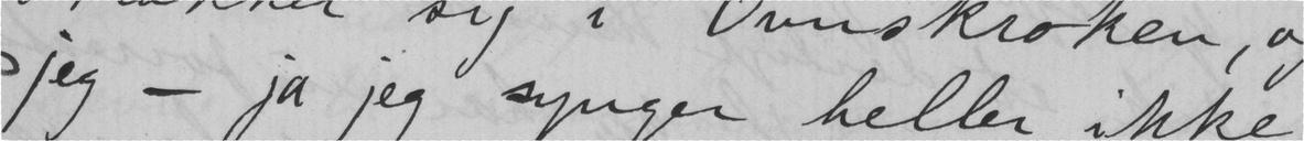jeg - ja jeg synger heller ikke
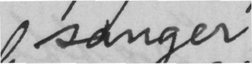sanger
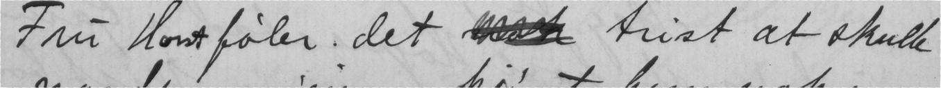Fru Horst føler det  trist at skulle
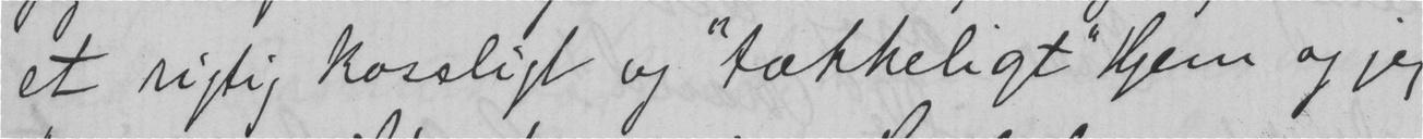et rigtig kossligt og "tækkeligt" Hjem og jeg
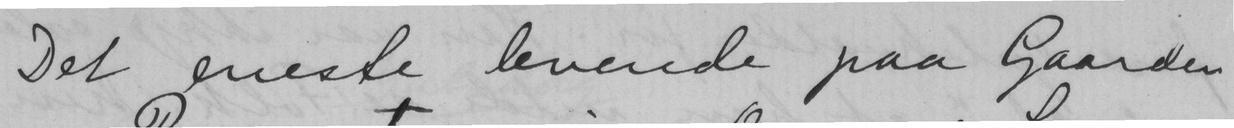Det eneste levende paa Gaarden
